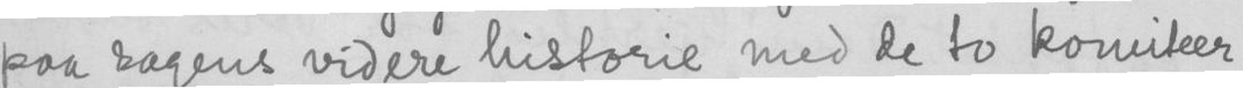paa sagens videre historie med de to komiteer
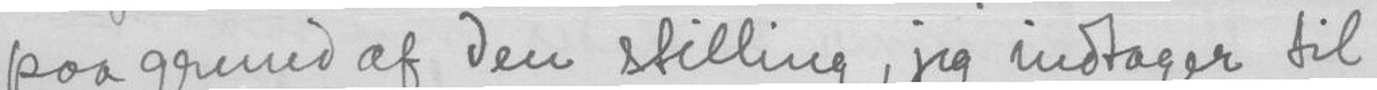paa grund af den stilling, jeg indtager til
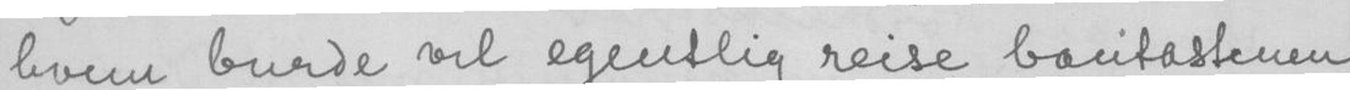hvem burde vel egentlig reise bautastenen
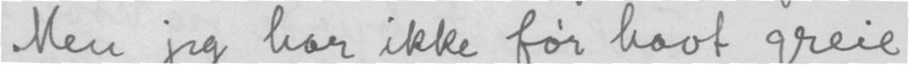Men jeg har ikke før havt greie
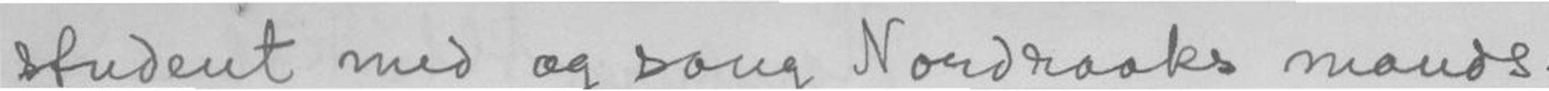student med og ang Nordraachs mands
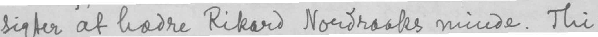sigter at hædre Rikard Nordraaks minde. Thi
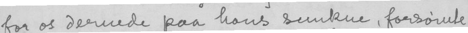for os dernede paa hans sunkne, forsømte
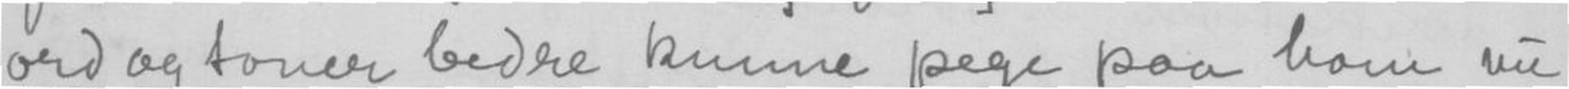ord og toner bedre kunne pege paa ham nu
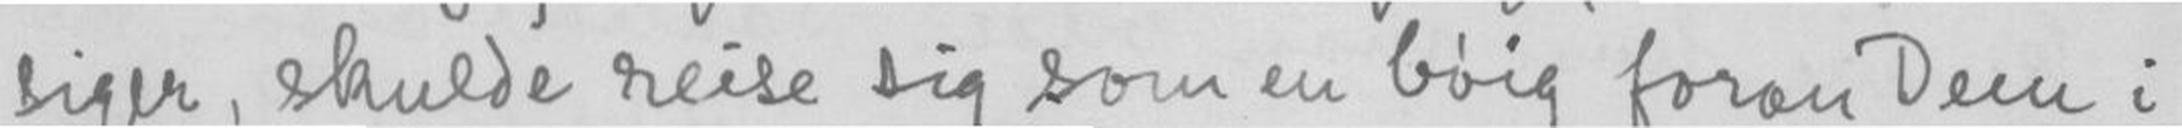siger, skulde reise sig som en bøig foran Dem i
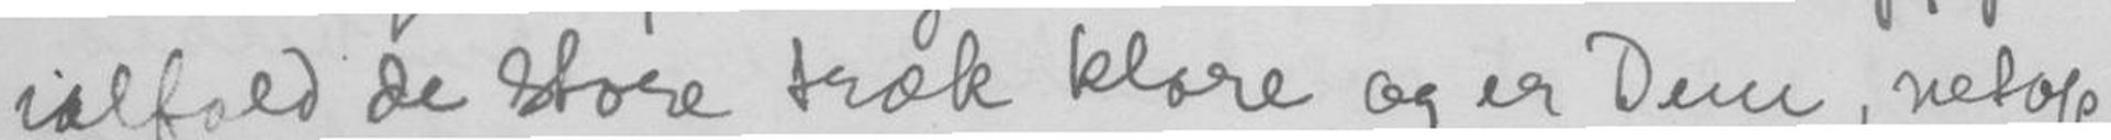ialfald de store træk klare og er Dem, netop
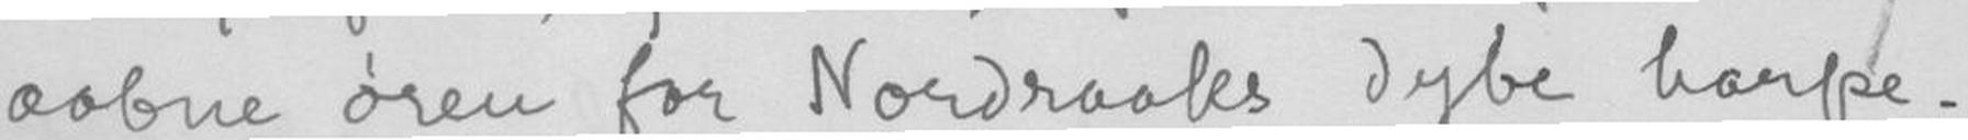aabne øren for Nordraaks dybe harpe.
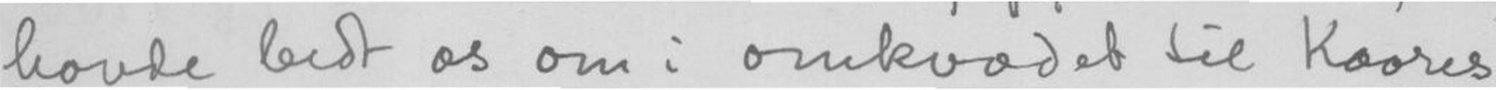havde bedt os om i omkvædet til Kaares
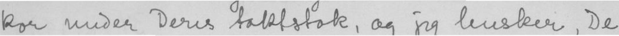kor under Deres taktstok, og jeg husker, De
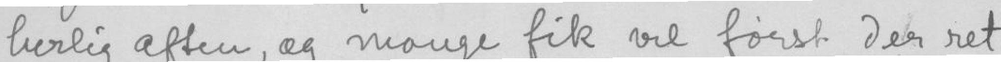herlig aften, og mange fik vel først Der ret
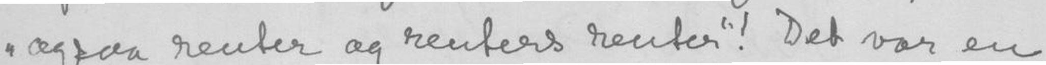ogsaa renter og rentes renter! Det var en
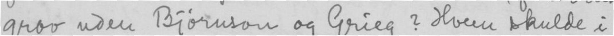grav uden Bjørnson og Grieg? Hvem skulde i
